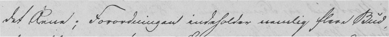det Rene. Forordningen indeholder nemlig flere Bud,
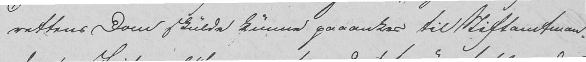rettens Dom skulde kunne paaankes til Stiftamtman-
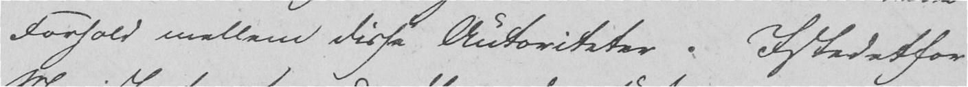Forhold mellem disse Autoriteter. Istedetfor
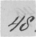48.
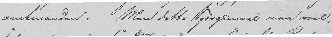amtmanden. Men dette Spørgsmaal maa vel,
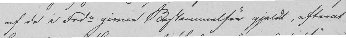af de i Frdn givne Bestemmelser gjaldt, efterat
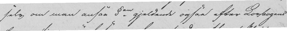selv om man ansaa §en gjeldende ogsaa efter Lovbogens
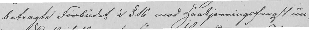betragte Forbudet i § 16 mod Haakjerringsfangst un-
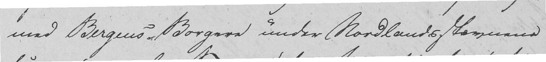med Bergens Borgere under Nordlandsstævnene
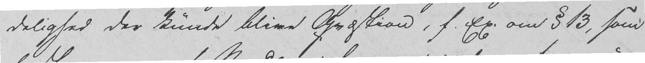delighed der kunde blive Qvæstion, f. Ex. om § 13, som
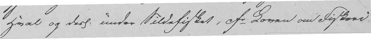Hval og desl. under Sildefisket, cfr Loven om Fiskeri
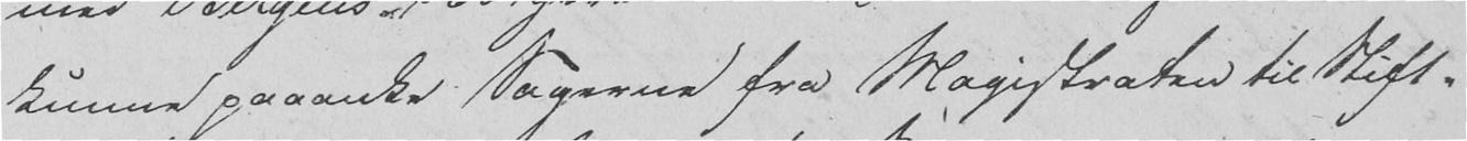kunne paaanke Sagerne fra Magistraten til Stift-
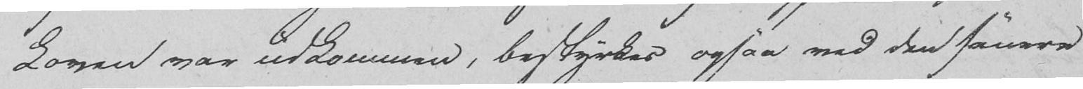Loven var udkommen, bestyrkes ogsaa med den senere
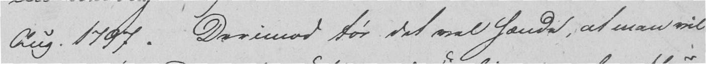Aug. 1797. Derimod tør det vel hænde, at man vil
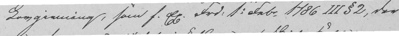Lovgivning, som f. Ex. Frd. 1. Febr. 1786 III § 2, der
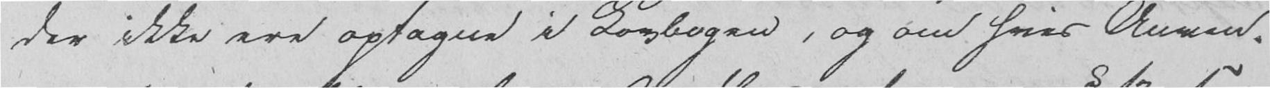der ikke ere optagne i Lovbogen, og om hvis Anven-
